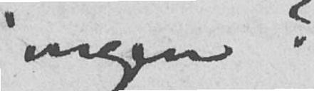ingen?
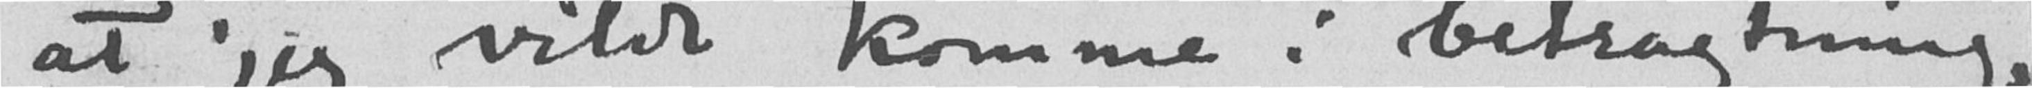at jeg vilde komme i betragtning,
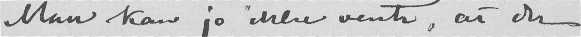Man kan jo ikke vente, at der
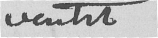ventet
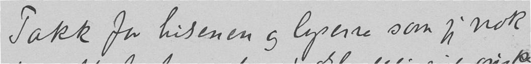Takk for hilsenen og lyserne som jeg nok
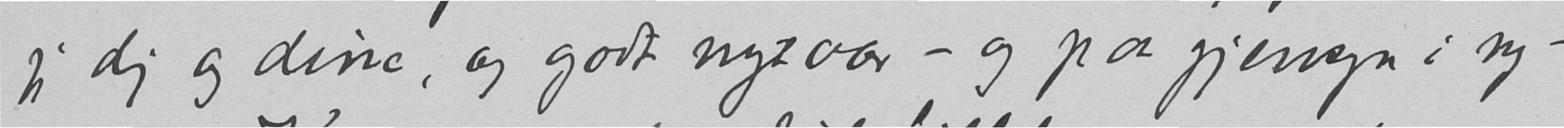jeg dig og dine, og godt nytaar – og paa gjensyn i ny –
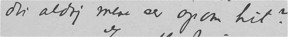du aldrig mere ser opom hit?
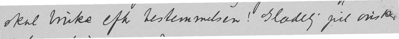skal bruke efter bestemmelsen! Glædelig jul ønsker
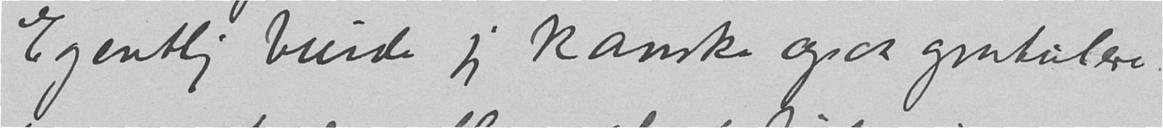Egentlig burde jeg kanske ogsaa gratulere
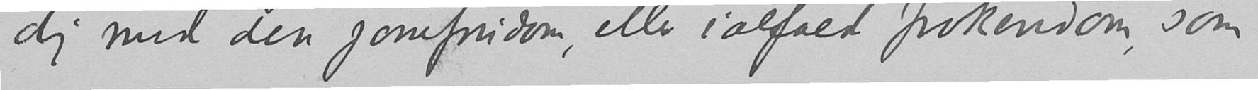dig med den jomfrudom, eller ialfald frøkendom, som
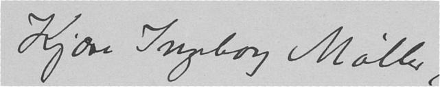Kjære Ingeborg Møller
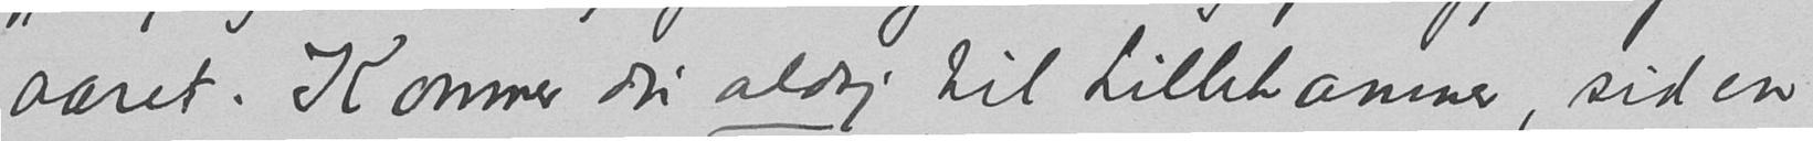aaret. Kommer du aldrig til Lillehammer, siden
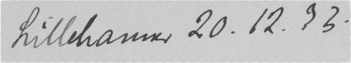Lillehammer 20.12.33.
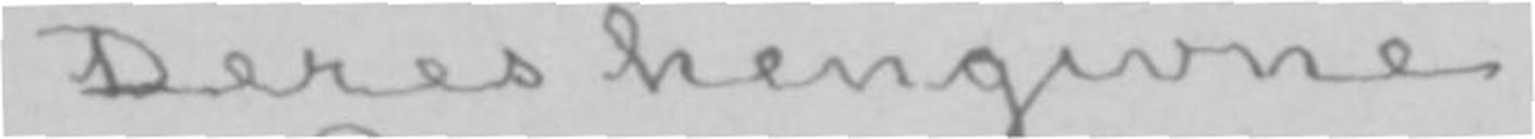Deres hengivne
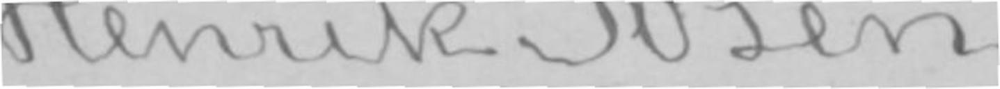Henrik Ibsen.
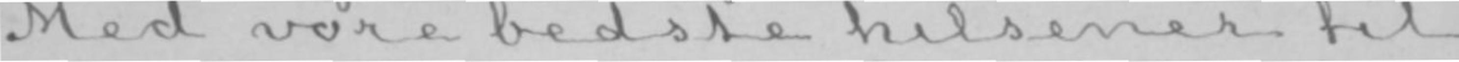Med vore bedste hilsener til
